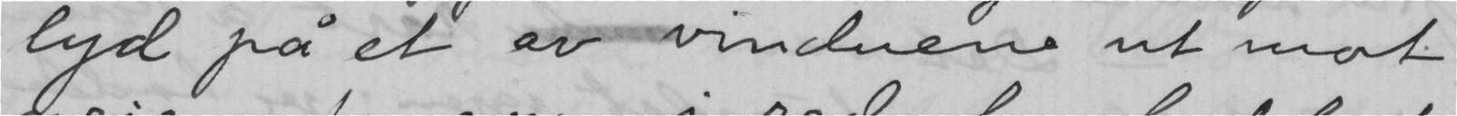lyd på et av vinduene ut mot
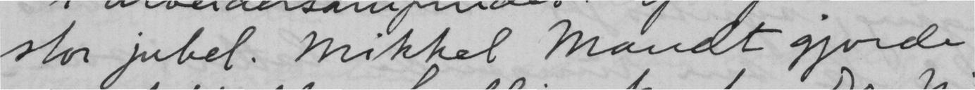stor jubel. Mikkel Mandt gjorde
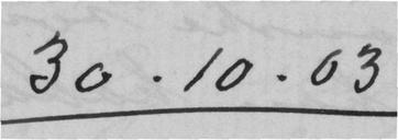30.10.03
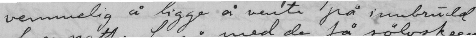vemmelig å ligge å vente på innbrudd
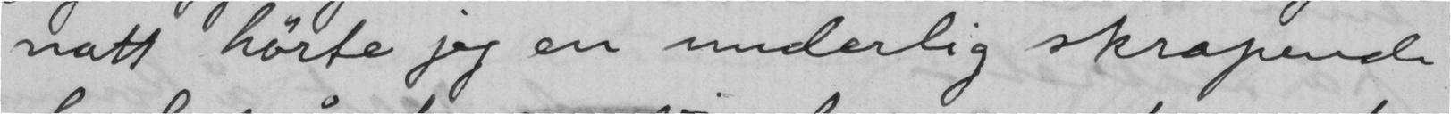natt hørte jeg en underlig skrapende
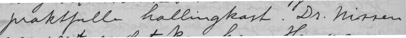praktfulle hallingkast. Dr. Nissen
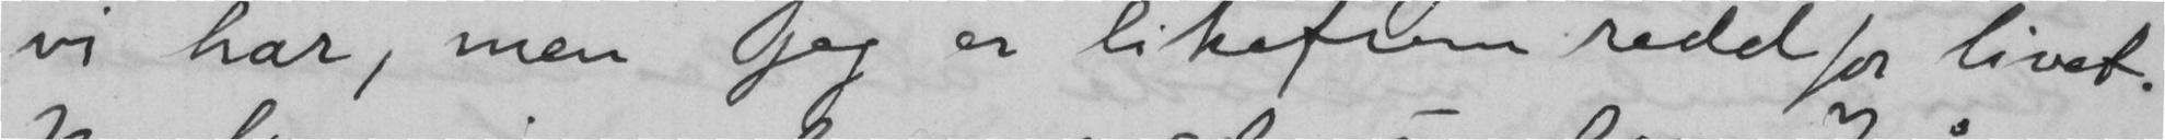vi har, men jeg er likefrem redd for livet.
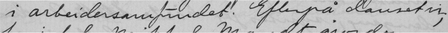i arbeidersamundet. Efterpå danset vi;
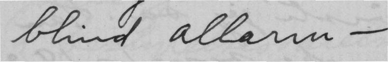blind allarm -
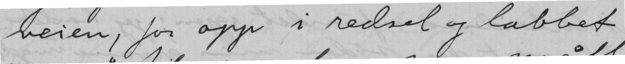veien, for opp i redsel og labbet
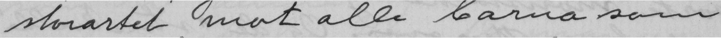storartet mot alle barna som
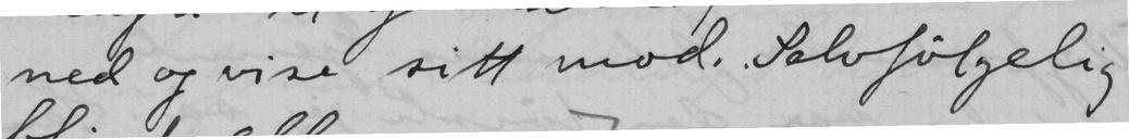ned og vise sitt mod. Selvfølgelig
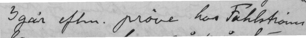Igår aften. prøve hos Fahlstrøms
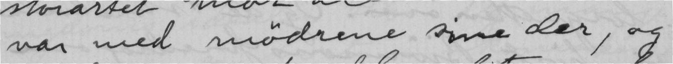var med mødrene sine der, og
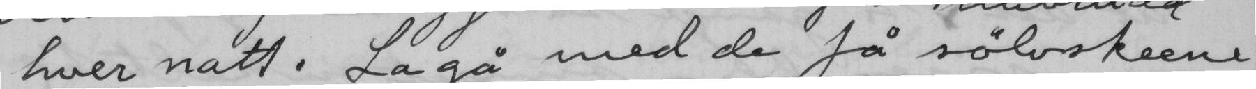hver natt. La gå med de få sølvskeene
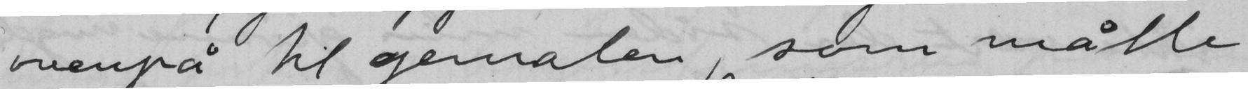ovenpå til gemalen, som måtte
